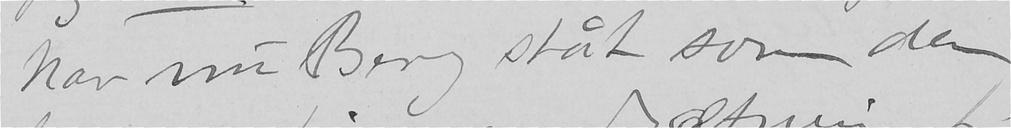har nu Berg ståt som den
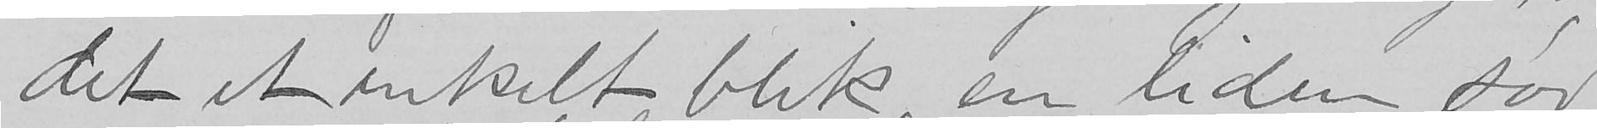det et enkelt blik, en liden sød
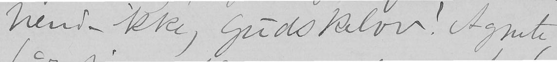hende ikke, gudskelov! Agnete
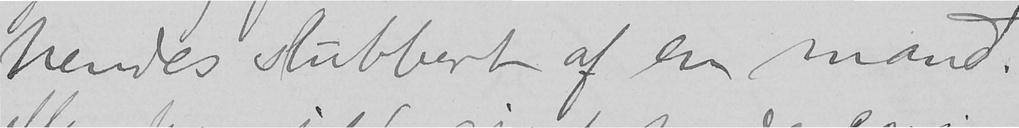hendes slubbert af en mand.
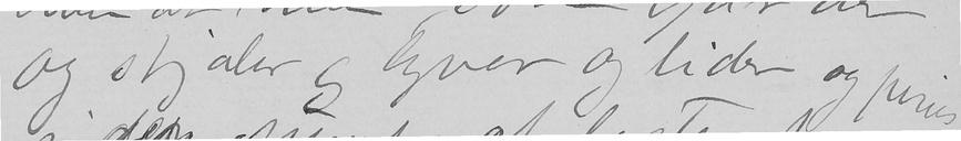og stjæler og lyver og lider og pines
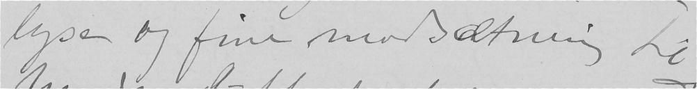lyse og fine modsætning til
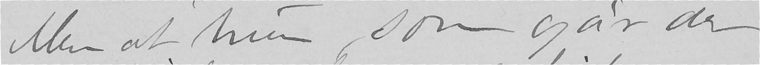Men at hun som går der
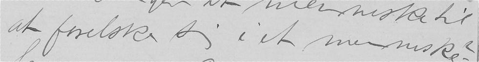at forelske sig i et menneske?
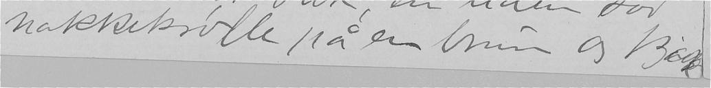nakkekrølle på en brun og kjæk
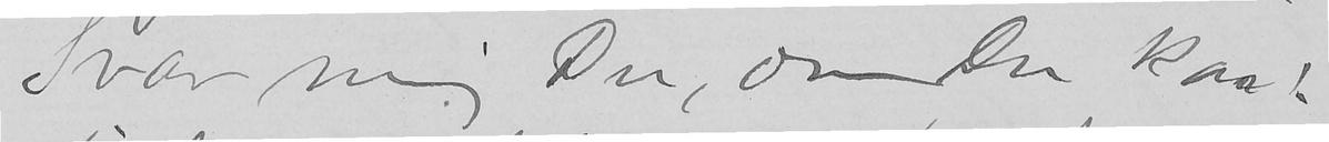Svar mig Du, om Du kan!
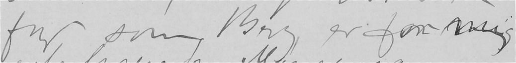fyr som Berg, er for mig
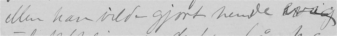Men han vilde gjort hende evig
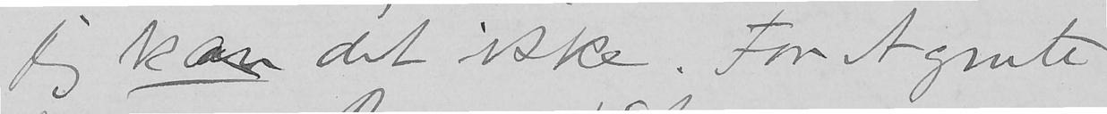Jeg kan det ikke. For Agnete
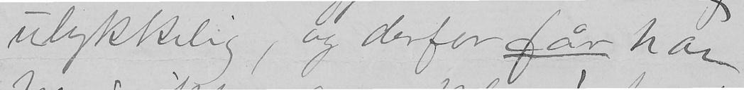ulykkelig, og derfor får han
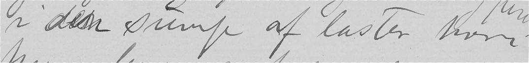i den sump af laster hvori
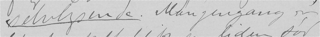selvlysende. Mangengang er
